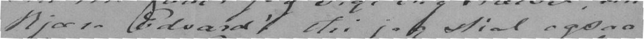kjære Edvard! thi jeg skal ogsaa
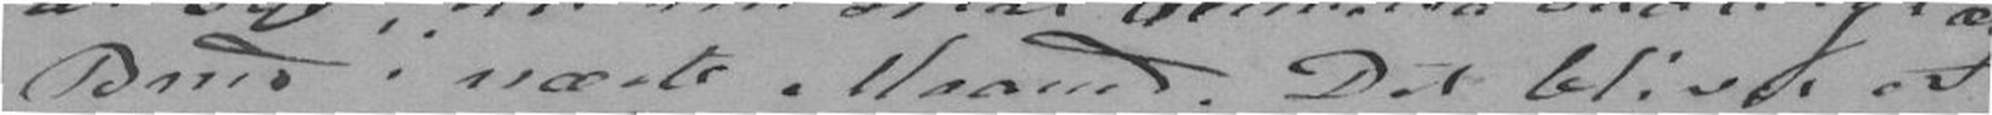Brud i næste Maaned. Det bliver et
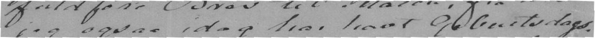jeg ogsaa idag har lovet Geburtsdags-
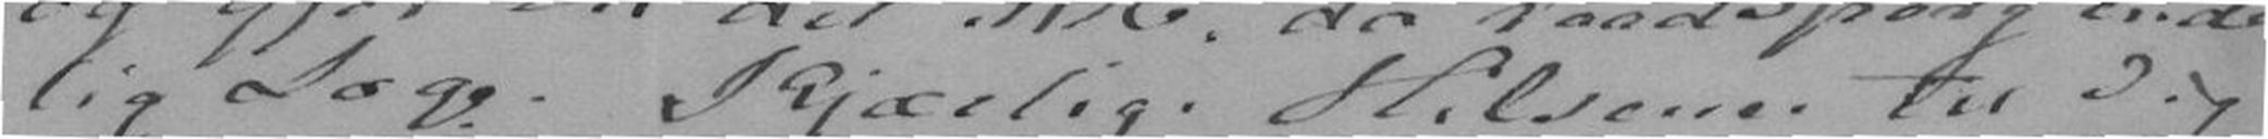lig Læge. Kjærlig Hilsener til Dig
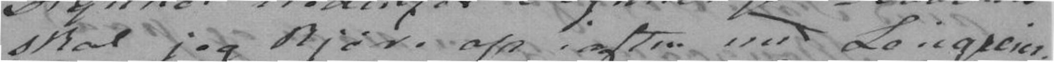skal jeg kjøre op i aften med Lengrein.
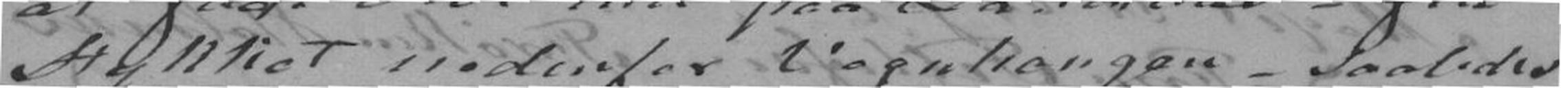Stykket nedenfor Vognhougen - saaledes
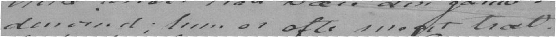denvind; hun er ofte meget træt.
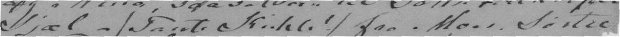Sjæl -/ Tante Kühle1/ fra Moer, Søstre
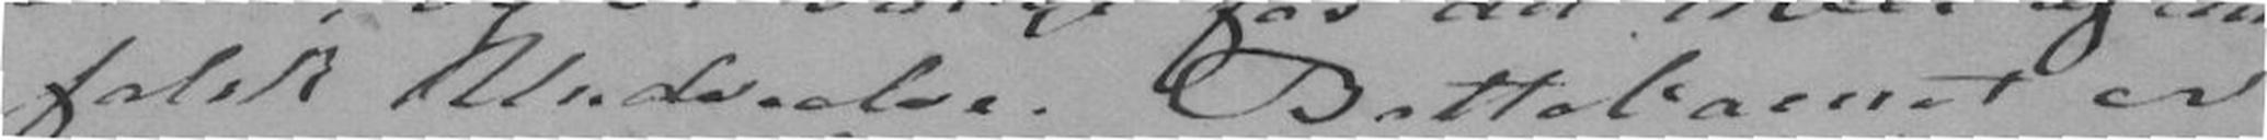falsk Undseelse. Bettebarnet er
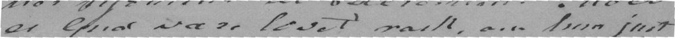er Gud være lovet rask, om hun just
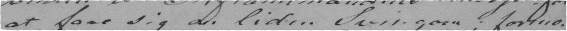at faae sig en liden Svingom; formo-
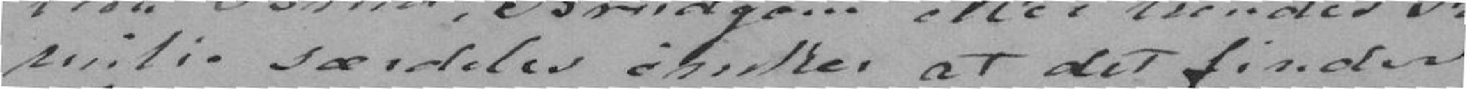milie særdeles ønsker at det finder
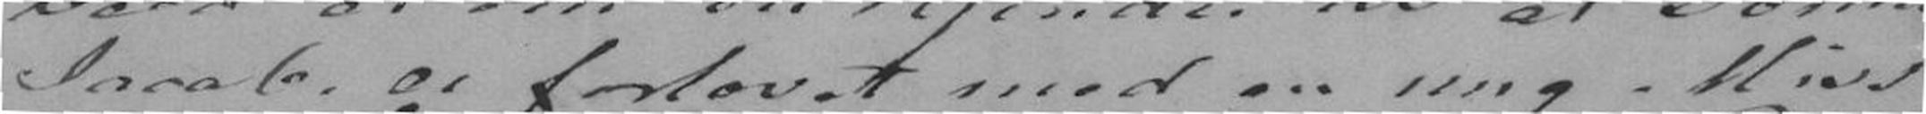Jacob, er forlovet med en ung Miss
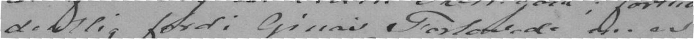dentlig fordi Ginas Forlovede nu er
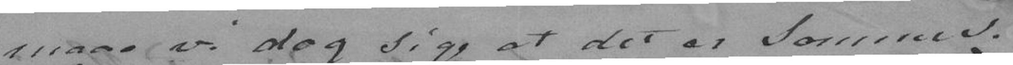maa vi dog sige at det er Sommer.
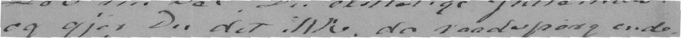og gjør Du det ikke, da raadspørg ende-
